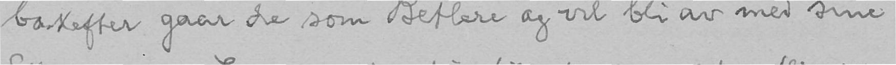bakefter gaar de som Betlere og vil bli av med sine
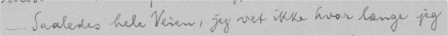- Saaledes hele Veien, jeg vet ikke hvor længe jeg
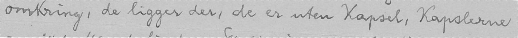omkring, de ligger der, de er uten Kapsel, Kapslerne
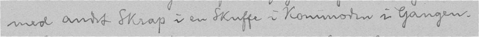med andet Skrap i en Skuffe i Kommoden i Gangen.
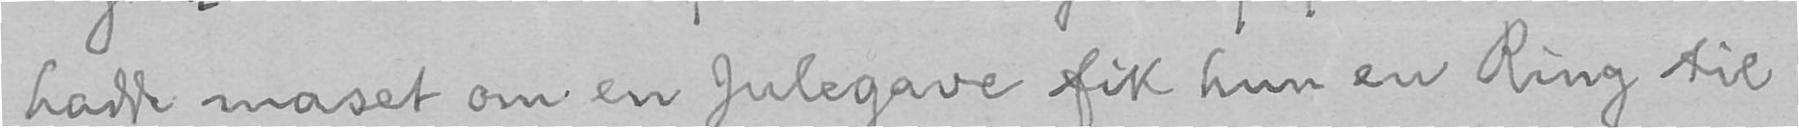hadde maset om en Julegave fik hun en Ring til
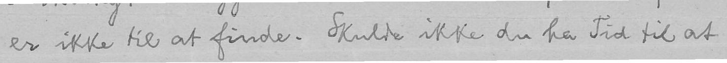er ikke til at finde. Skulde ikke du ha Tid til at
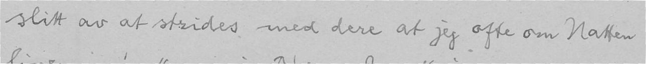slitt av at strides med dere at jeg ofte om Natten
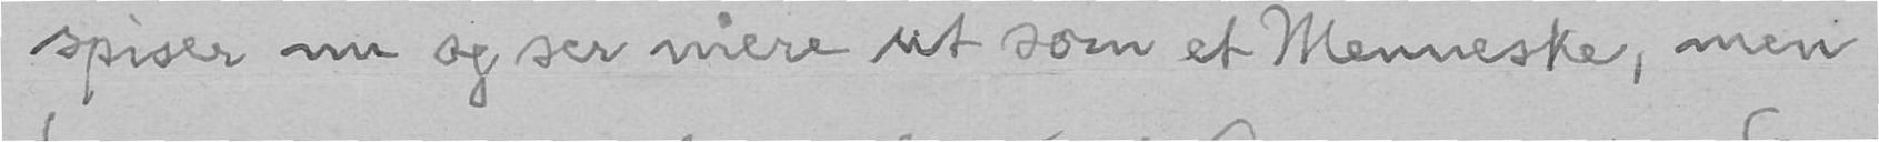spiser nu og ser mere ut som et Menneske, men
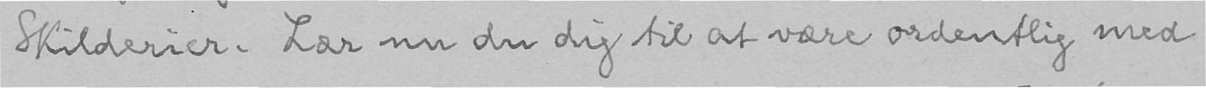Skilderier. Lær nu du dig til at være ordentlig med
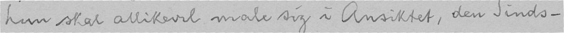hun skal allikevel male sig i Ansiktet, den Sinds-
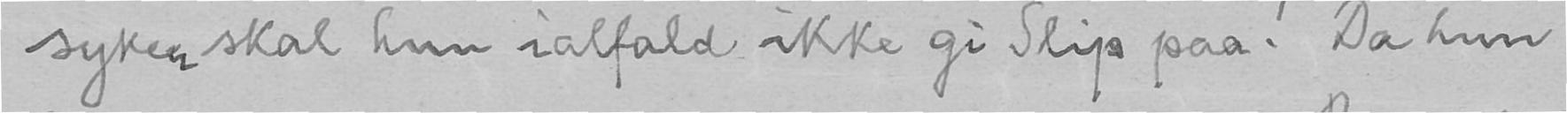syken skal hun ialfald ikke gi Slip paa! Da hun
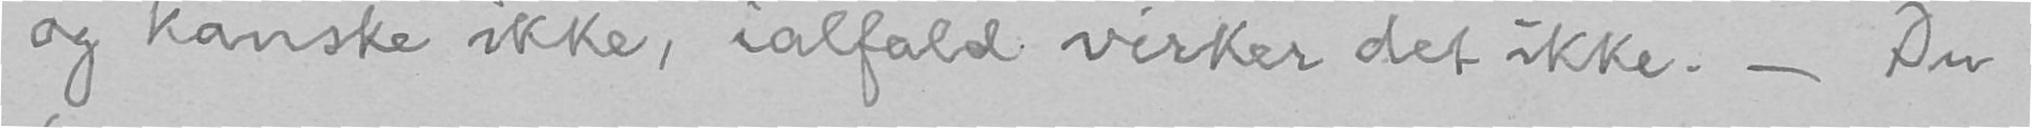og kanske ikke, ialfald virker det ikke. - Du
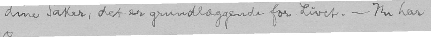dine Saker, det er grundlæggende for Livet. - Nu har
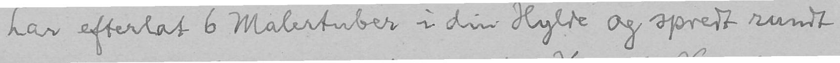har efterlat 6 Malertuber i din Hylde og spredt rundt
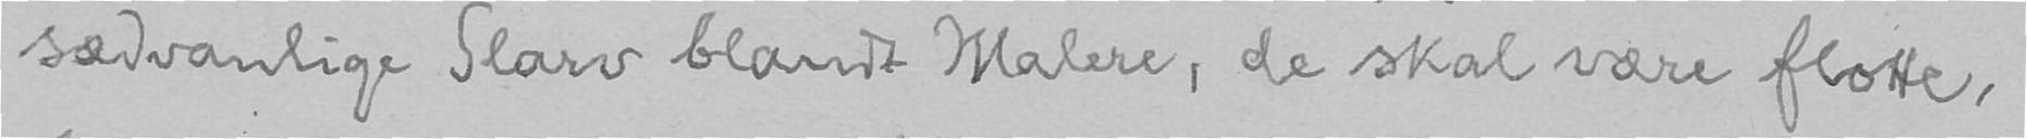sædvanlige Slarv blandt Malere, de skal være flotte,
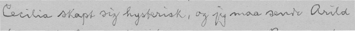Cecilia skapt sig hysterisk, og jeg maa sende Arild
